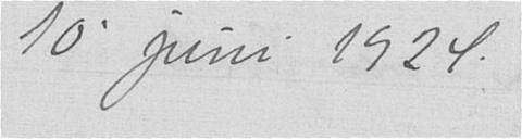10 . juni 1924.
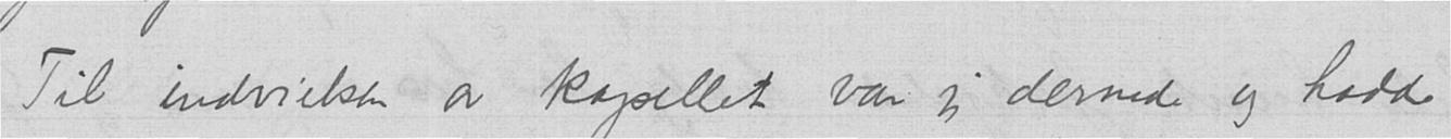Til indvielsen av kapellet var jeg dernede og hadde
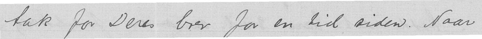tak for Deres brev for en tid siden. Naar
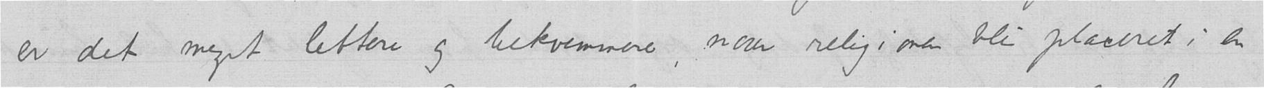er det meget lettere og bekvemmere, naar religionen blir placeret i en
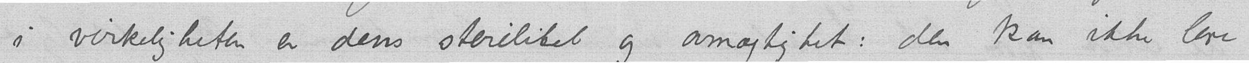i virkeligheten er dens sterilitet og avmægtighet: den kan ikke lære
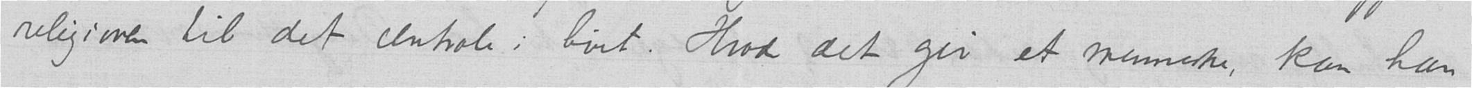religionen til det centrale i livet. Hvad det gir et menneske, kan han
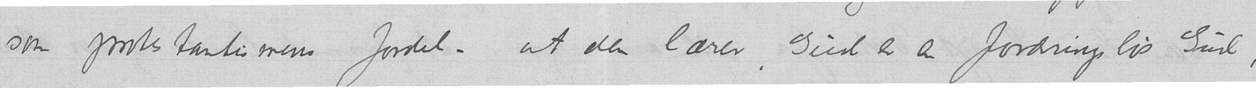som protestantismens fordel – at den lærer, Gud er en fordringsløs Gud,
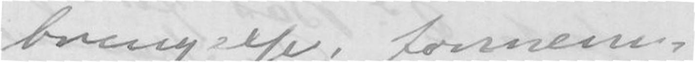bringelse, fornem-
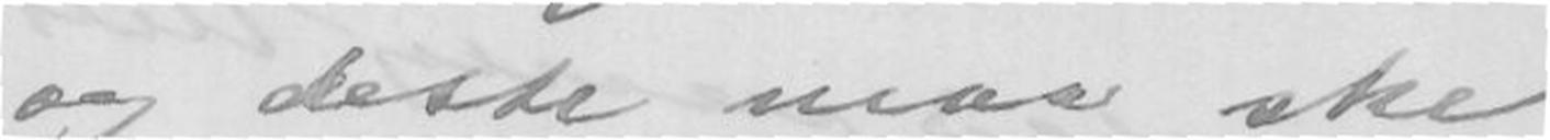og dette maa ske
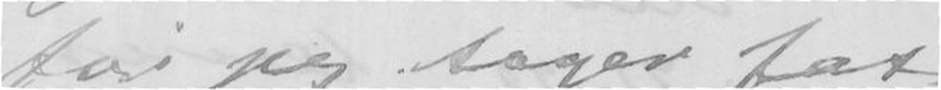før jeg tager fat
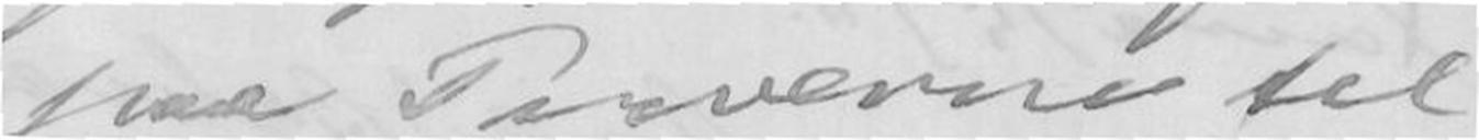paa Prøverne til
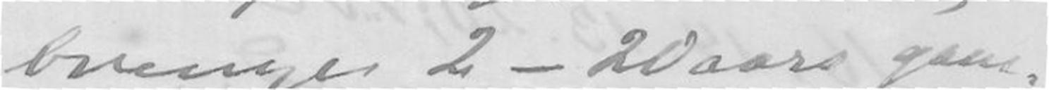bringe 2 - 20 aars gam-
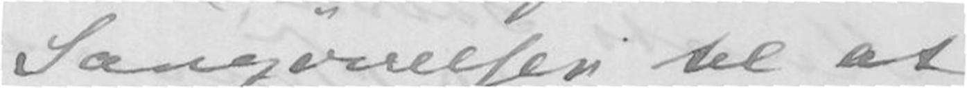Sangøvelser til at
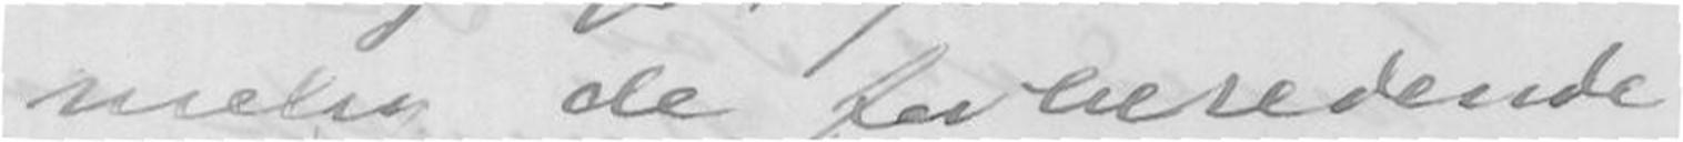melse de forberedende
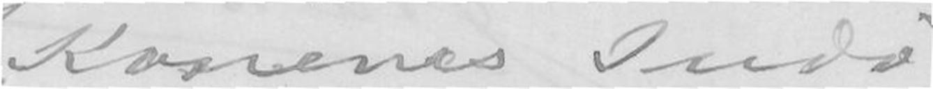Korenes Indø-
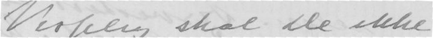Visselig skal De ikke
